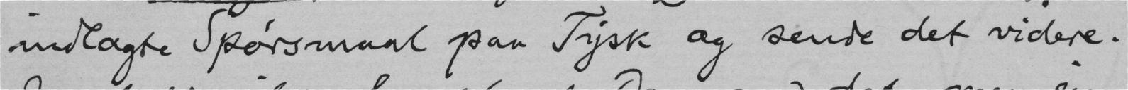indlagte Spørsmaal paa Tysk og sende det videre.
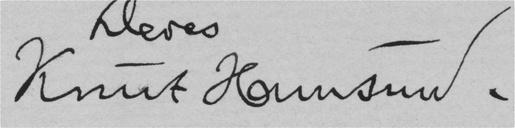Knut Hamsun.
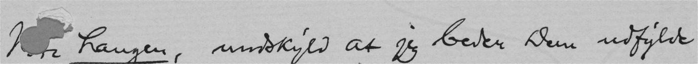Langen, undskyld at jeg beder Dem udfylde
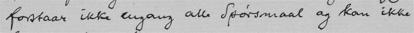forstaar ikke engang alle Spørsmaal og kan ikke
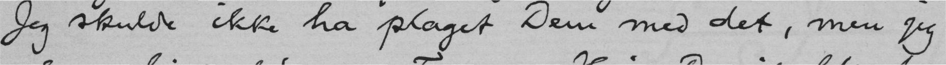Jeg skulde ikke ha plaget Dem med det, men jeg
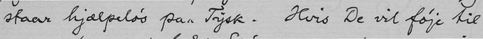staar hjælpeløs paa Tysk. Hvis De vil føje til
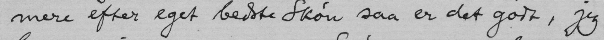mere efter eget bedste Skøn saa er det godt, jeg
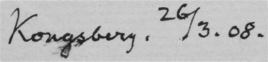Kongsberg. 26/3.08.
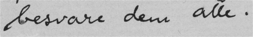besvare dem alle.
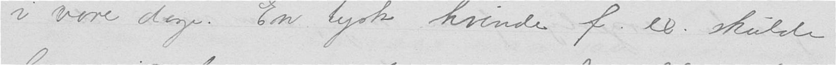i vore dage. En tysk kvinde f.ex. skulde
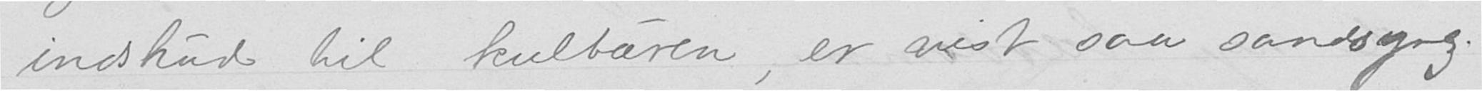inskud til kulturen, er vist saa sandsynlig
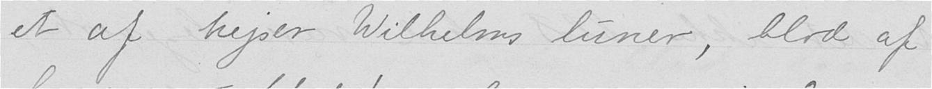et af kejser Wilhelms luner, blod af
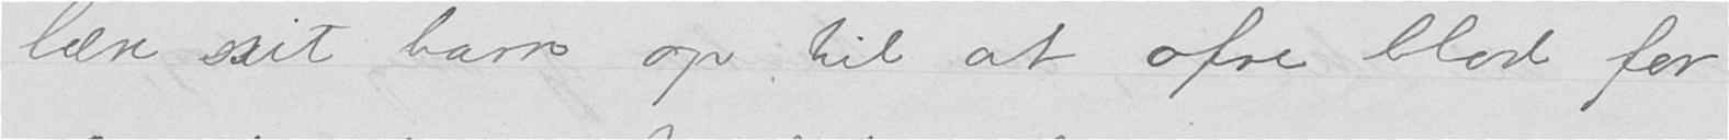lære sit barn op til at ofre blod for
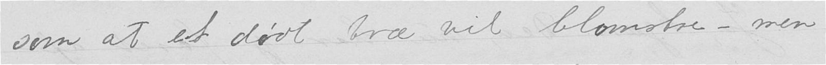som at et dødt træ vil blomstre - men
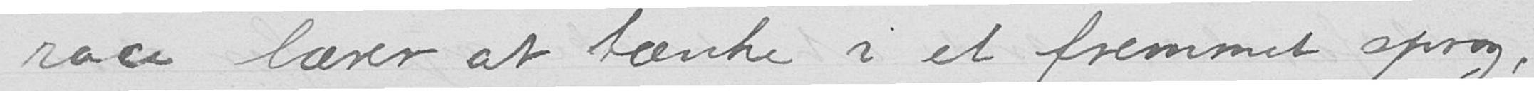race lærer at tænke i et fremmed sprog,
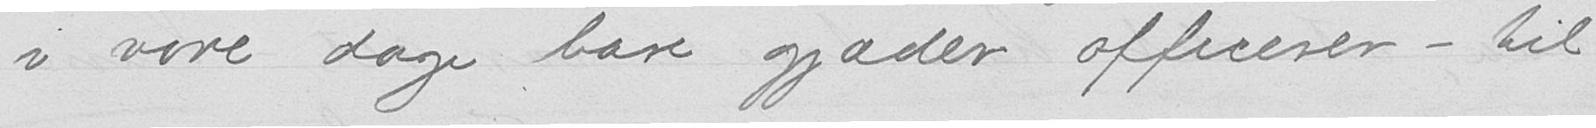i vore dage bare gjelder officerer - til
